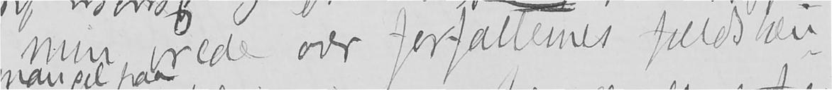min vrede over forfatternes fuldsten-
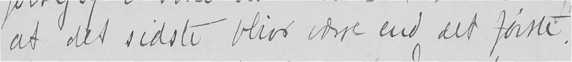at det sidste bliver værre end det første.
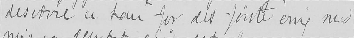desværre er han for det første enig med
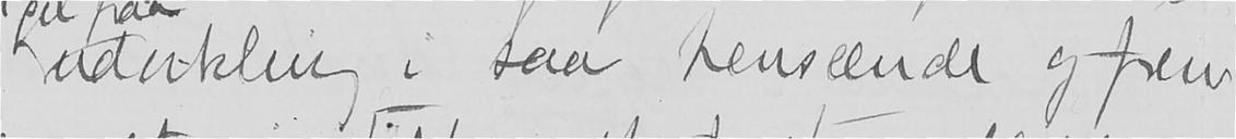udvikling i saa henseende og frem-
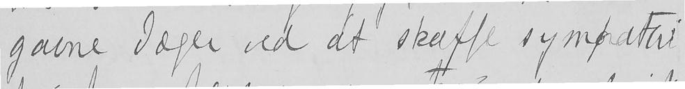gavne Jæger ved at skaffe sympathi
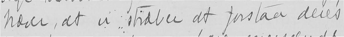hæver, at vi stræber at forstaa deres
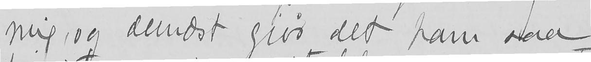mig, og dernæst gjør det ham saa
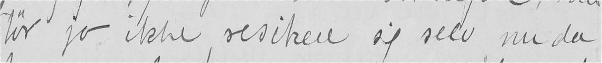tør jo ikke resikere sig selv, nu da
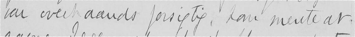var overhaands forsigtig, han mente at
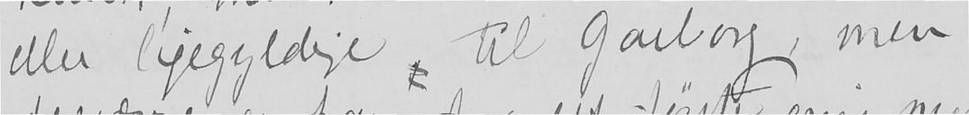eller ligegyldige til Garborg, men
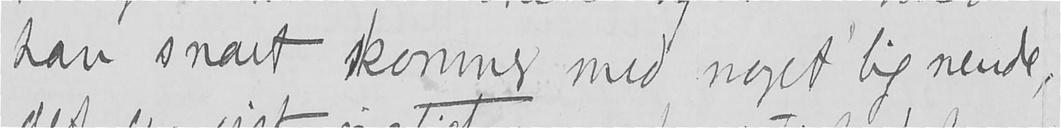han snart kommer med noget lignende,
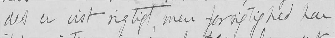det er vist rigtigt, men forsigtighed har
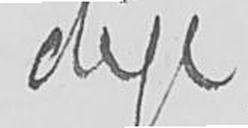dige
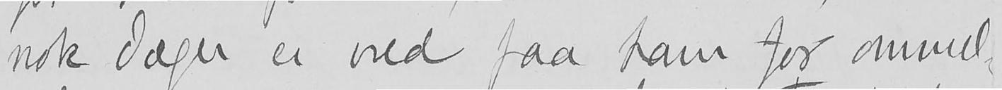nok Jæger er vred paa ham for anmel-
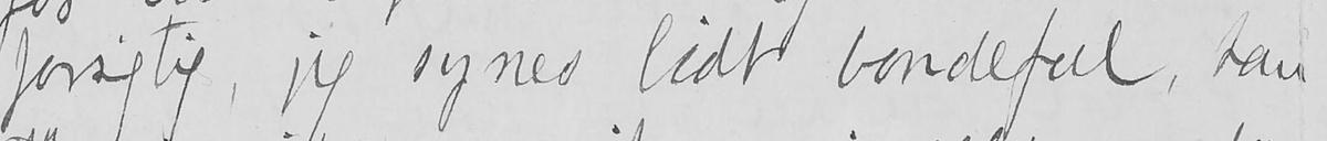forsigtig, jeg synes lidt bondeful, han
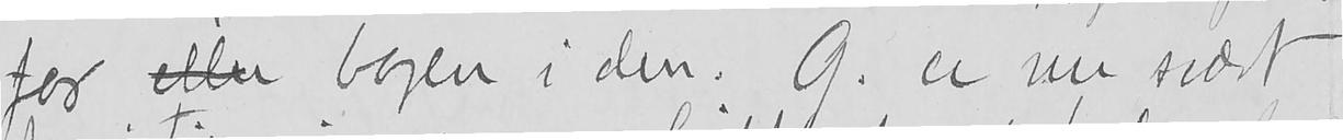for eller bogen i den. G. er nu svært
